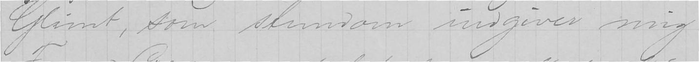Glimt, som stundom indgiver mig
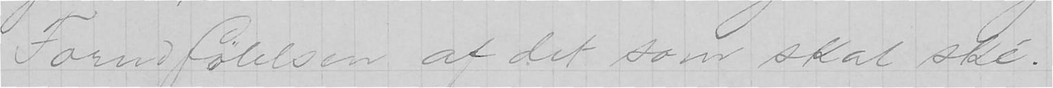Forudfølelsen af det som skal ské.
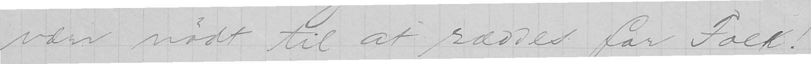være nødt til at ræddes for Folk!
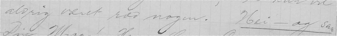aldrig været ræd nogen. Nei - og saa
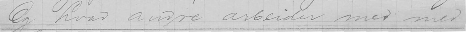Og hvad andre arbeider med med
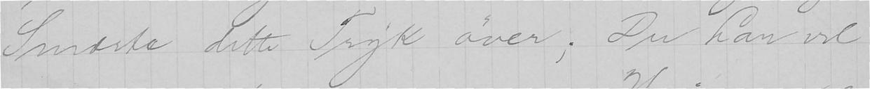Smærte dette Tryk øver; Du har vel
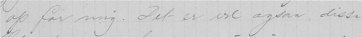op for mig. Det er vel ogsaa disse
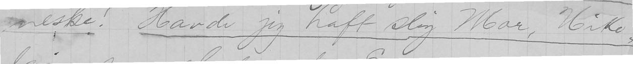neske! Havde jeg haft slig Mor, Niko-
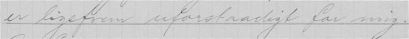er ligefrem uforstaaeligt for mig.
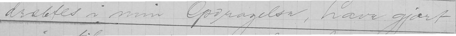dræbtes i min Opdragelse, have gjort
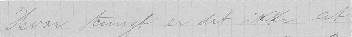Hvor tungt er det ikke at
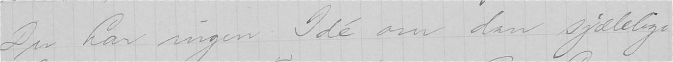Du har ingen Idé om den sjælelige
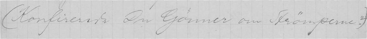(Konfirerede Du Gønner om Strømperne?)
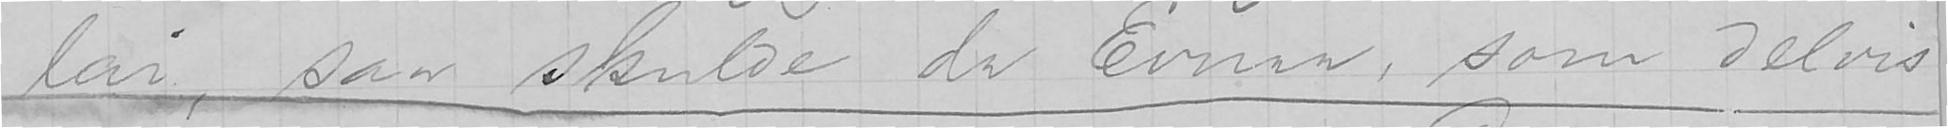lai, saa skulde da Evnen, som delvis
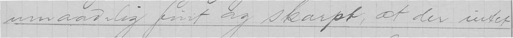umaadelig fint og skarpt, at der intet
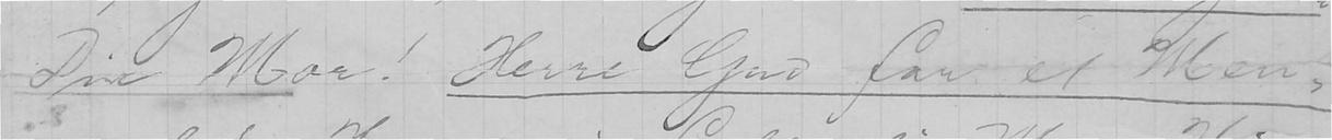Din Mor! Herre Gud for et Men-
